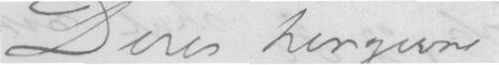Deres hengivne
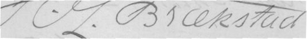H.L. Brækstad
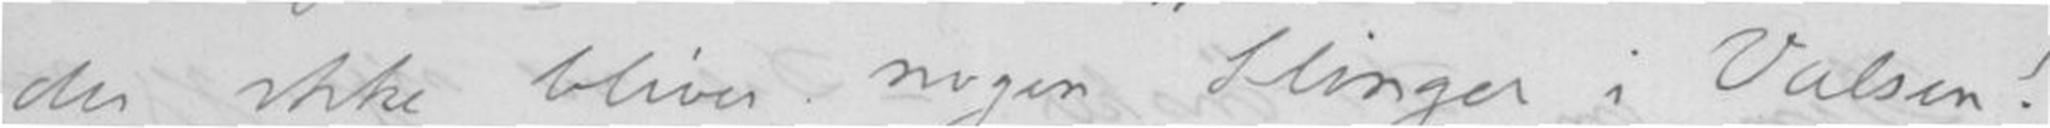der ikke bliver nogen "Slinger i Valsen!"
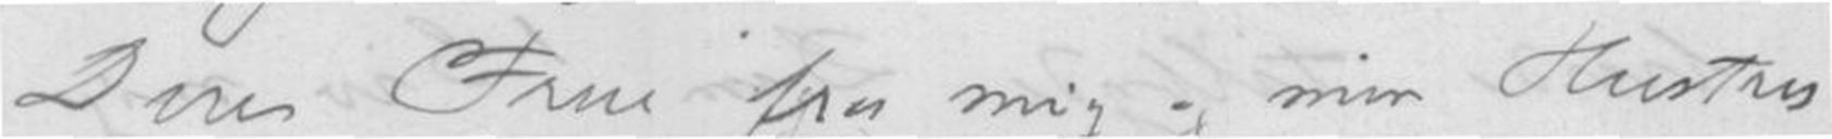Deres Frue fra mig og min Hustru
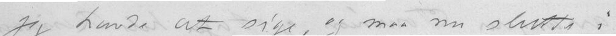jeg havde at sige, og maa nu slutte i
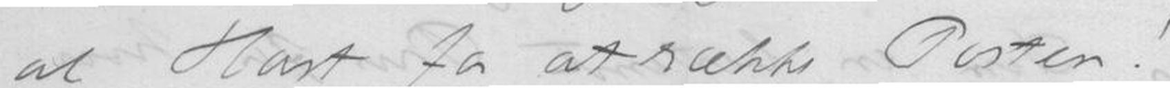al Hast for at række Posten! -
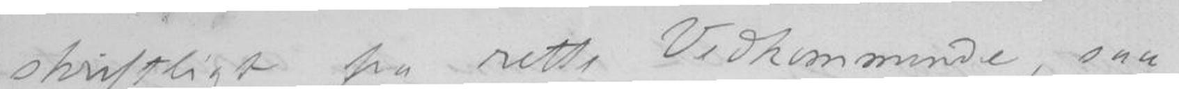skriftlig fra rette Vedkommende, saa
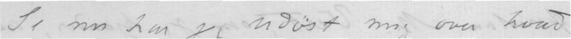Se nu har jeg udøst mig over hvad
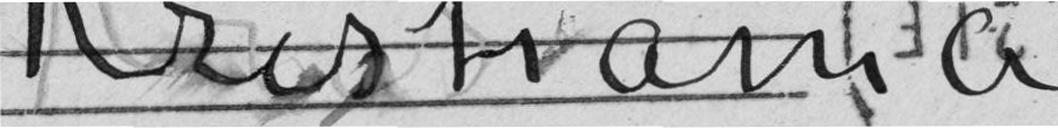Kristiania
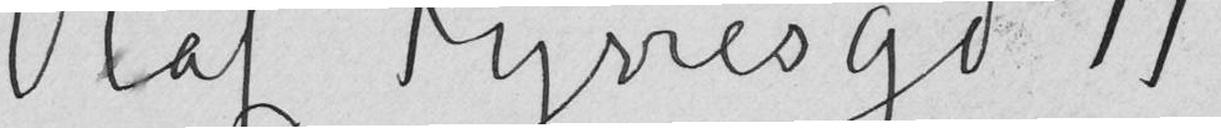Olaf Kyrresgd 11
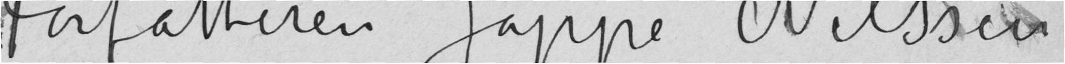Forfatteren Jappe Nilssen
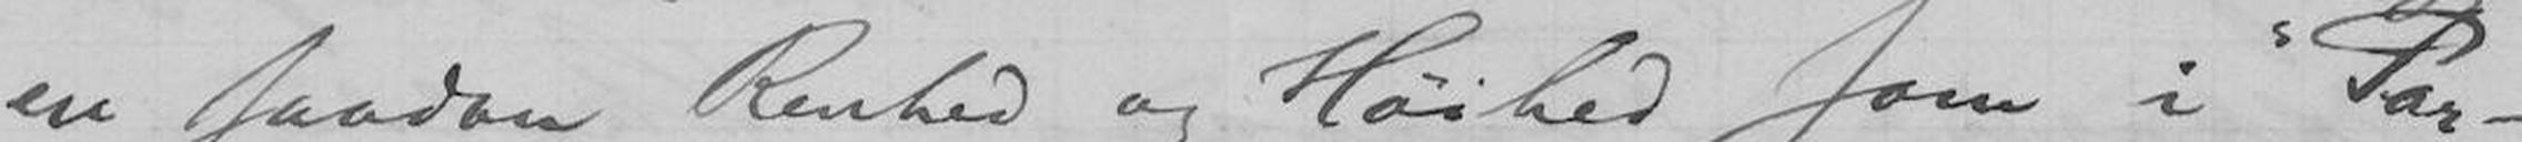en saadan Renhed og Høihed som i "Par-
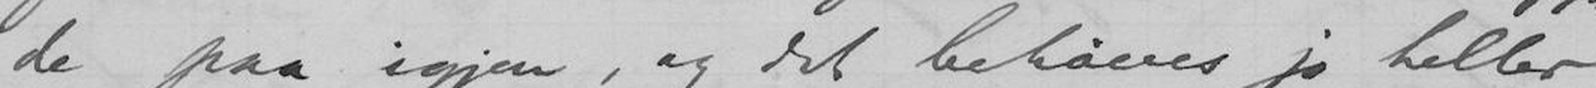de paa igjen, og det behøves jo heller
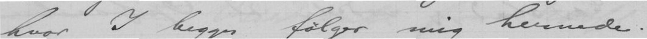hvor I begge følger mig hernede.
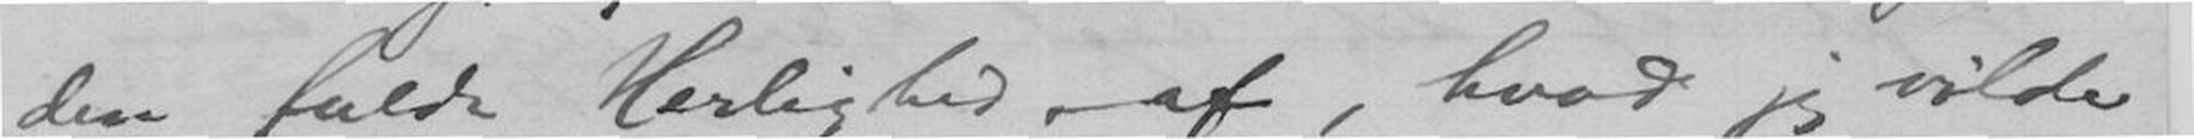den fulde Herlighed af, hvad jeg vilde
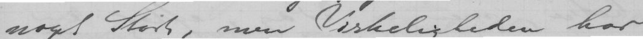noget Stort, men Virkeligheden har
